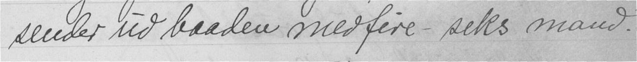sender ud baaden medfire-seks mand.
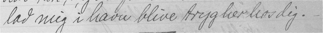lad mig i havn blive tryg her hos dig. -
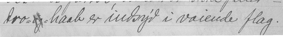tro-og-haab er indsy'd i vaiende flag.
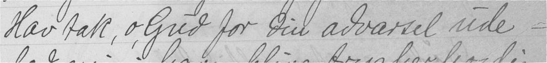Hav tak, o, Gud for din advarsel ude -
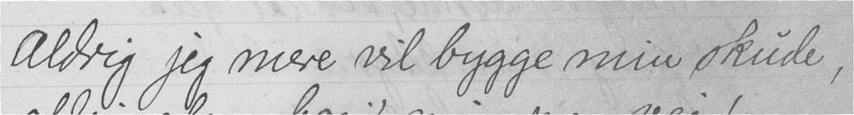aldrig jeg mere vil bygge min skude,
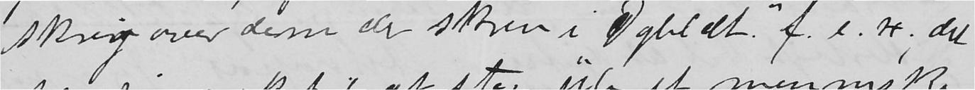skrig over dem de skrev i "Dgbldt" f.e.x. det
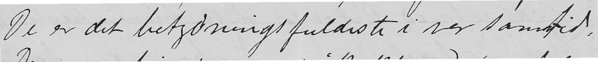De er det betydningsfuldeste i vor samtid.
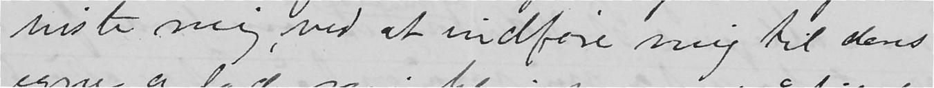viste mig, ved at indføre mig til deres
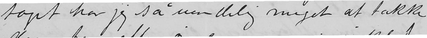taget har jeg så uendelig meget at takke
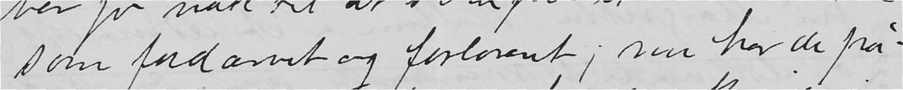som fordærvet og forlorent; nu har de på-
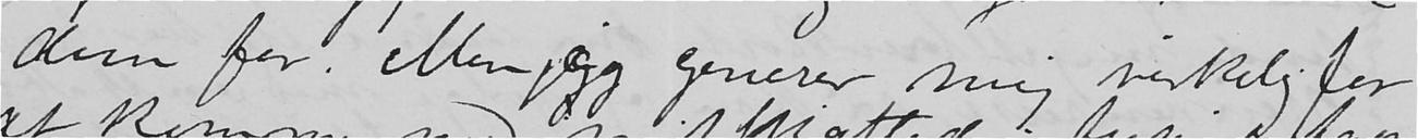dem for. Men jeg generer mig virkelig for
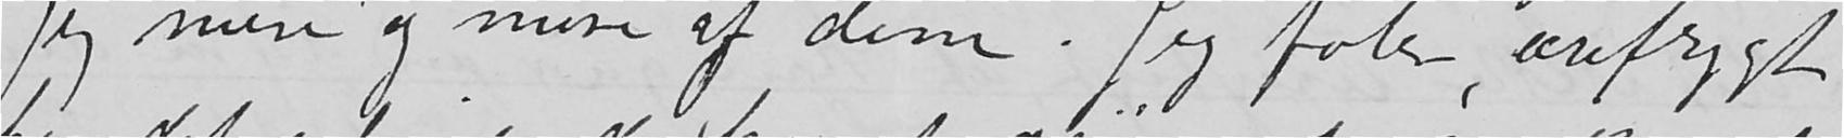jeg mere og mere af dem. Jeg føler ærefrygt
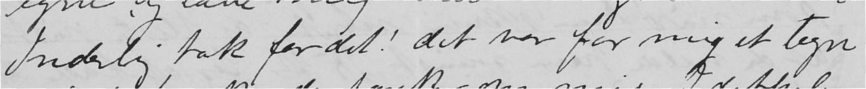Inderlig tak for det! det var for mig et tegn
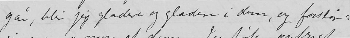går, blir jeg gladere og gladere i dem, og forstår
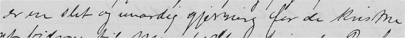er en slet og uværdig gjerning for de kristne
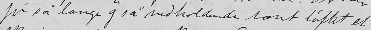jo så længe og så vedholdende været løftet et
